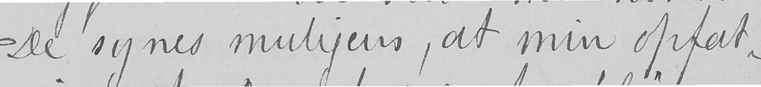De synes muligens, at min opfat-
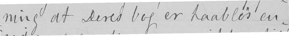ning af Deres bog er haabløs en-
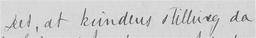Det, at kvindens stilling da
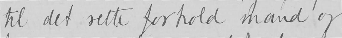til det rette forhold mand og
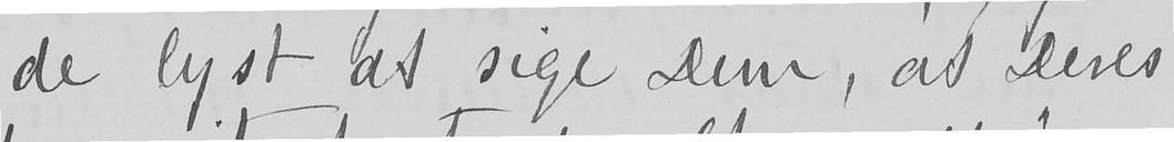de lyst at sige Dem, at Deres
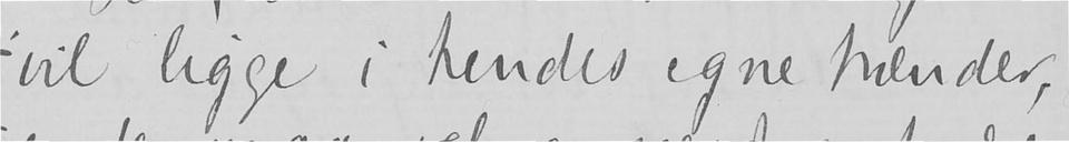vil ligge i hendes egne hænder,
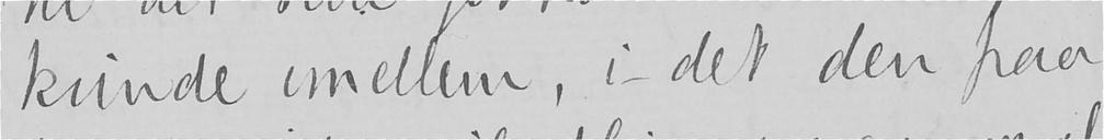kvinde imellem, i det den paa
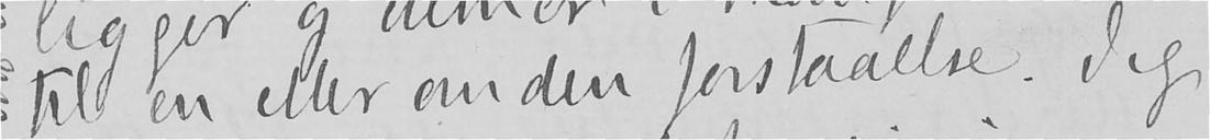til en eller anden forstaaelse. Jeg
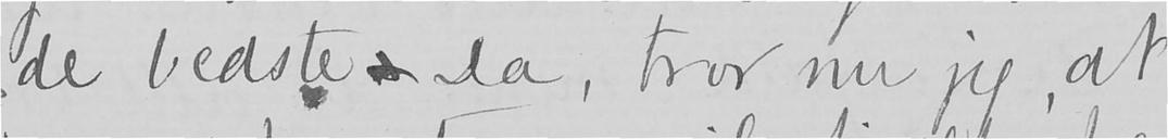de bedste. Da, tror nu jeg, at
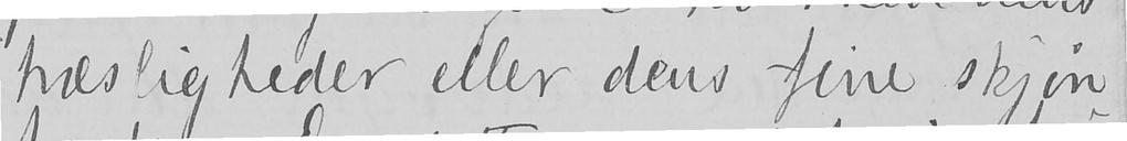hæsligheder eller dens fine skjøn-
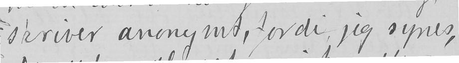skriver anonymt, fordi, jeg synes,
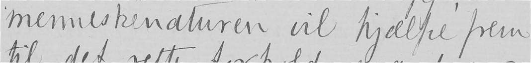menneskenaturen vil hjælpe frem
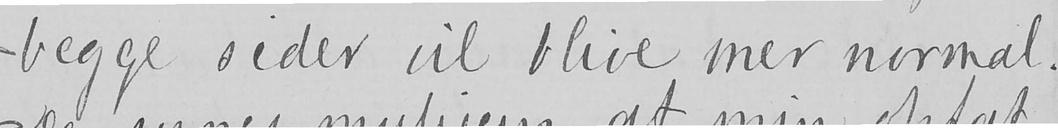begge sider vil blive mer normal.
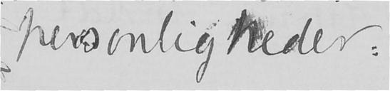personligheder.
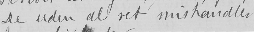De uden al ret mishandler
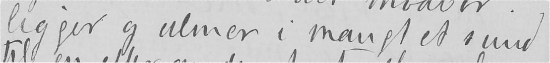ligger og ulmer i mangt et sind
